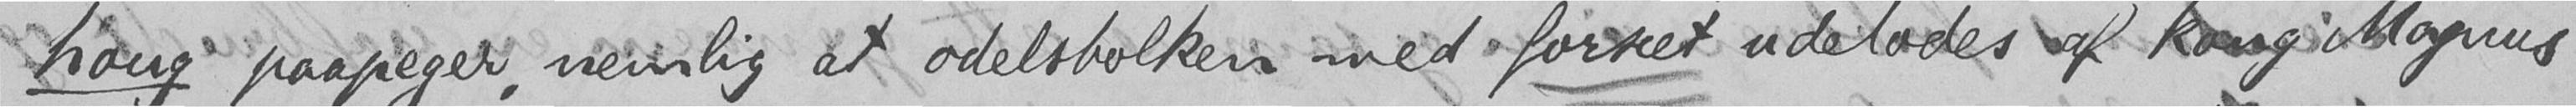houg paapeger, nemlig at odelsbolken med forset udelades af kong Magnus
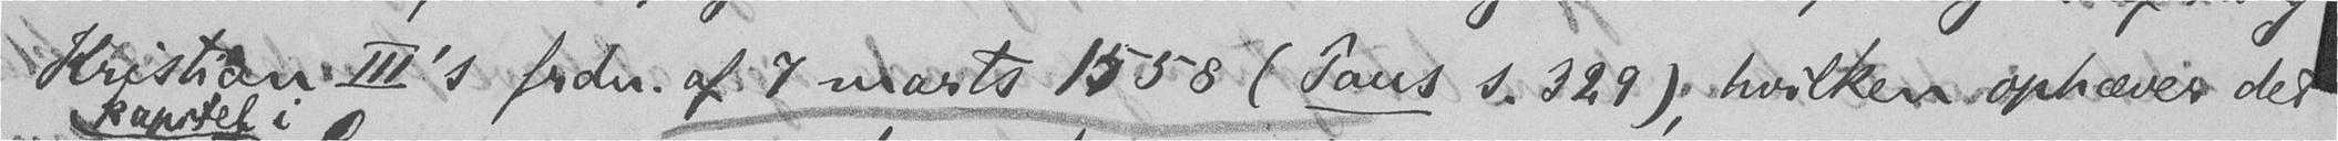Kristian III's frdn. af 7 marts 1558 (Paus s. 329), hvilken ophæver det
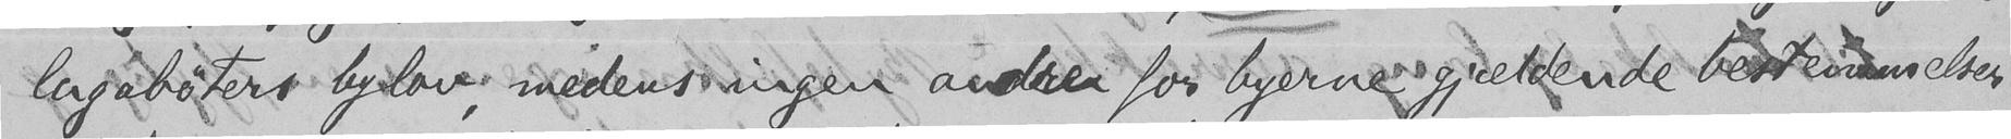lagabøters bylov, medens ingen andre for byerne gjældende bestemmelser
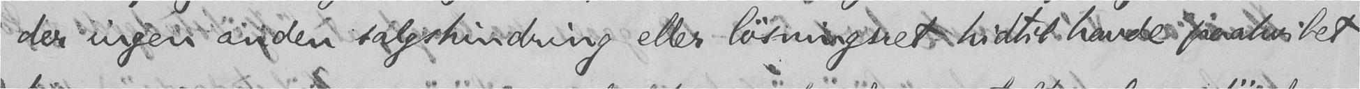der ingen anden salgshindring eller løsningsret hidtil havde paahvilet
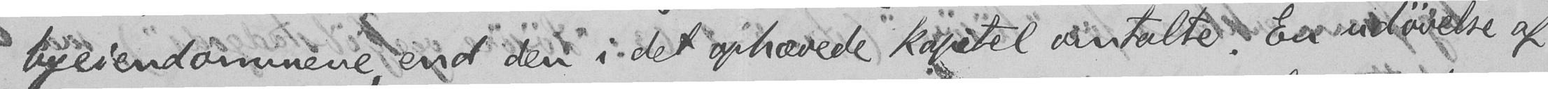byeiendommene, end den i det ophævede kapitel omtalte. En udøvelse af
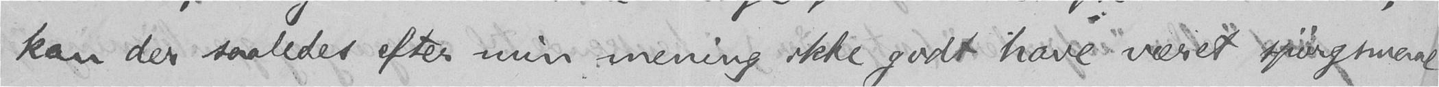kan der saaledes efter min mening ikke godt have været spørgsmaal
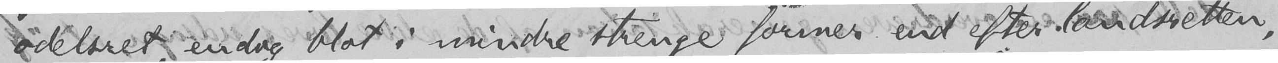odelsret, endog blot i mindre strenge former end efter landsretten,
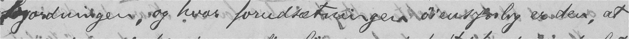byordningen, og hvor forudsætningen øiensynlig er den, at
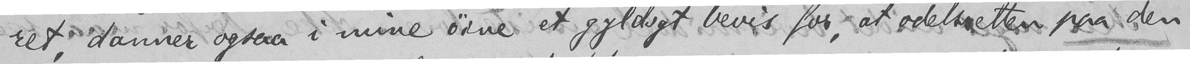ret, danner ogsaa i mine øine et gyldigt bevis for, at odelsretten paa den
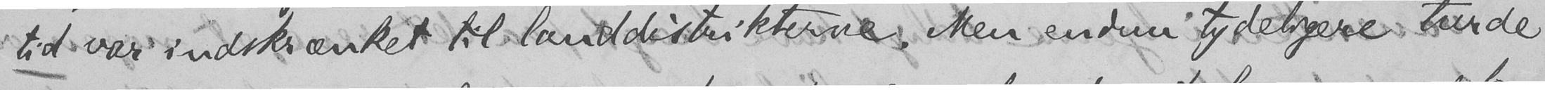tid var indskrænket til landdistrikterne. Men endnu tydeligere turde
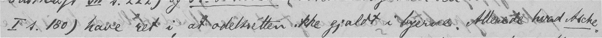I s. 180) have ret i, at odelsretten ikke gjaldt i byerne. Allerede hvad Asche-
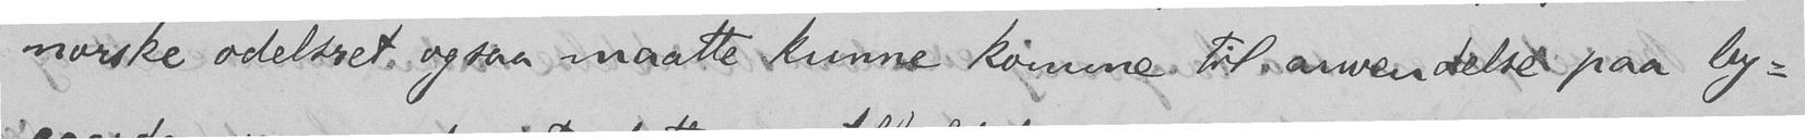norske odelsret ogsaa maatte kunne komme til anvendelse paa by-
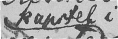kapitel i
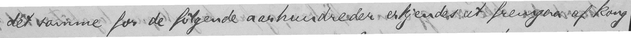det samme for de følgende aarhundreder erkjendes at fremgaa af kong
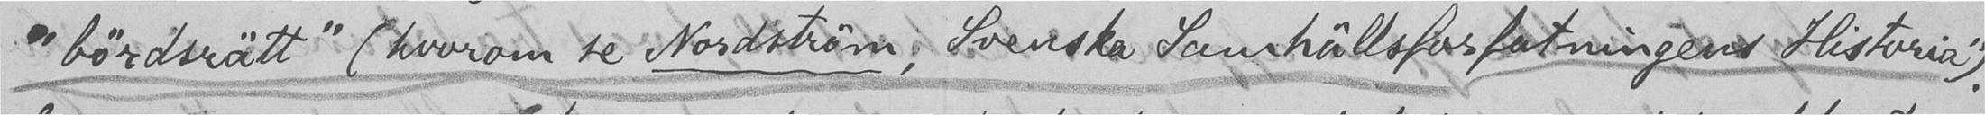"bördsrätt" (hvorom se Nordström, Svenske Samhällsforfatningens Historia").
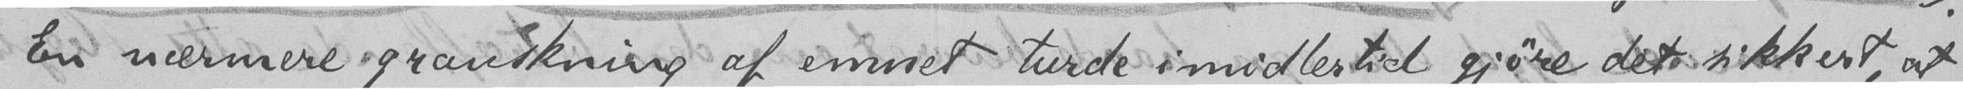En nærmere granskning af emnet turde imidlertid gjøre det sikkert, at
